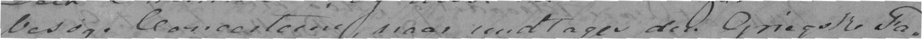besøge Concerterne, naar undtages den Griegske Fa-
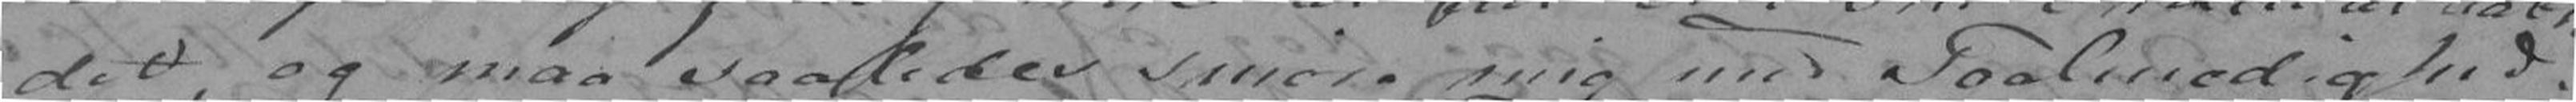det, og maa saaledes smøre mig med Taalmodighed.
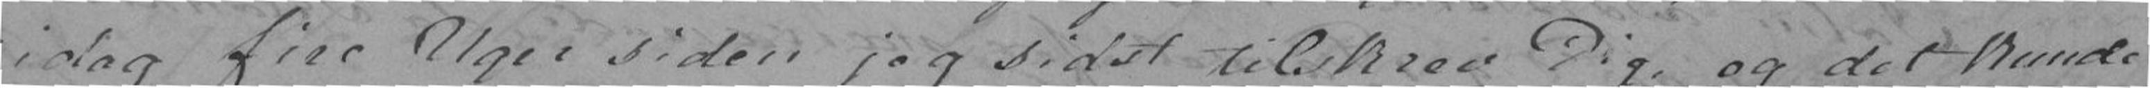idag fire uger siden jeg sidst tilskrev Dig, og det kunde
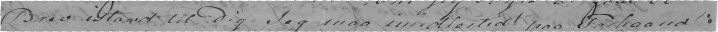Brev istand til Dig. Jeg maa imidlertidt paa Forhaand
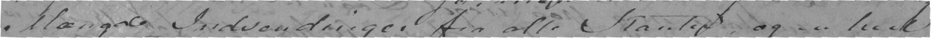Mangde Indvendinger fra alle Kanter, og en heel
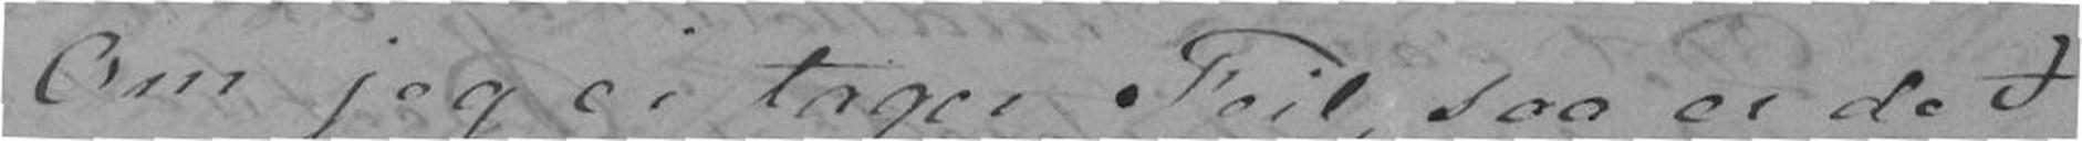Om jeg ei tager Feil saa er det
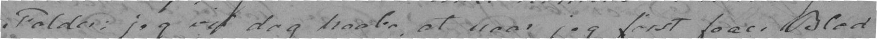Folde, jeg vil dog haabe, at naar jeg først faaer Blod
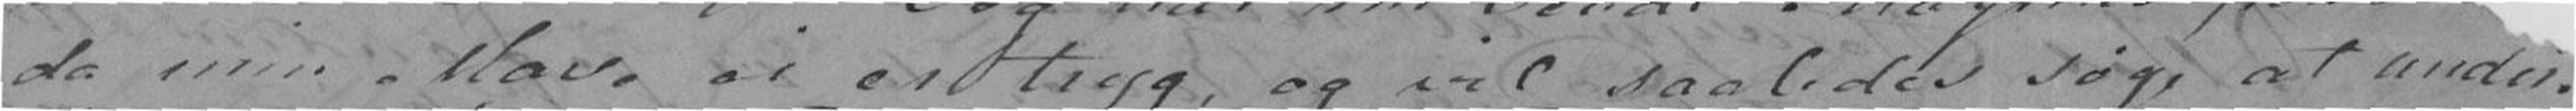da min Mave ei er tryg, og vil saaledes søge at under-
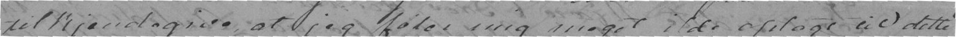tilkjendegive at jeg føler mig meget ilde oplagt til dette
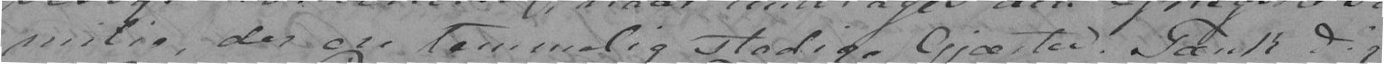milie, der ere temmelig stadige Gjæster. Tænk Dig
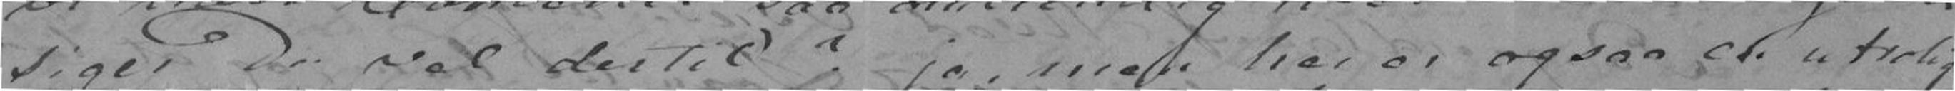siger Du vel dertil? ja, men har ei ogsaa en utrolig
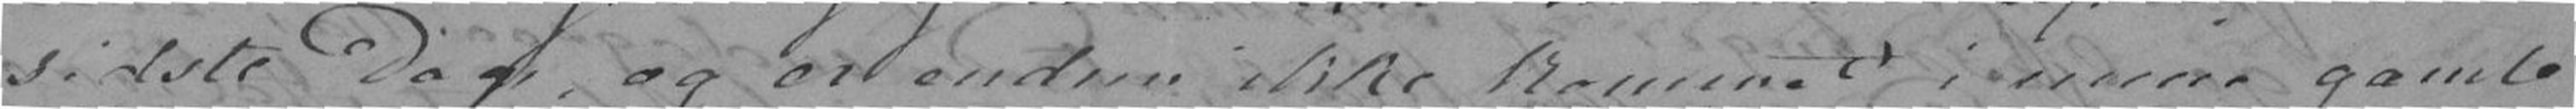sidste Dage, Og er endnu ikke kommet i mine gamle
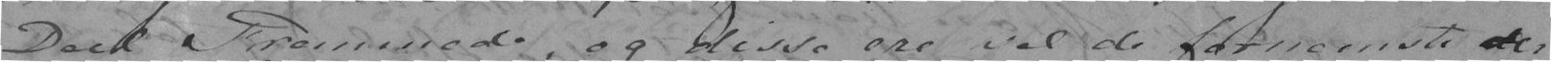Deel Fremmende, og disse ere vel de fornemste der
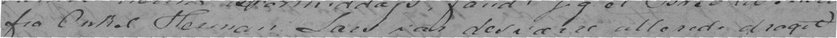fra Onkel Herman. Lars var desværre allerede draget
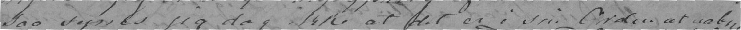saa synes jeg dog ikke at det er i sin Orden, at aabne
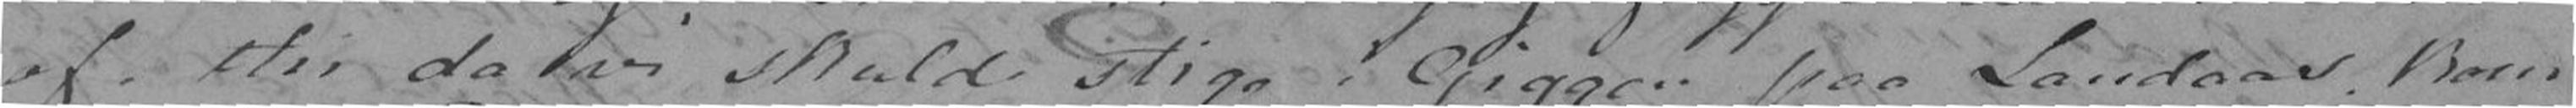af, thi da vi skulde stige i Giggen paa Landaas kom
